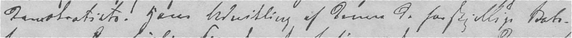Demokratiets. Hans Udvikling af denne de forskjellige Stats-
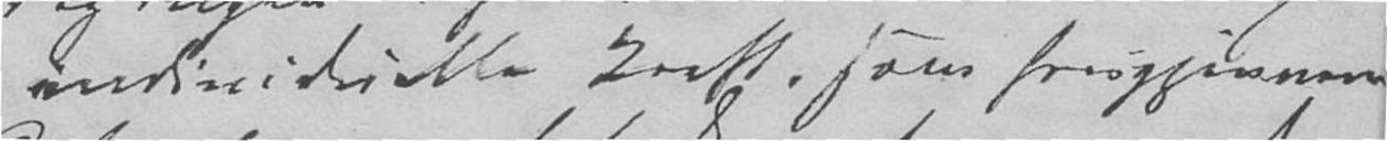individuelle Kraft, som herigjennem
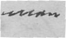man
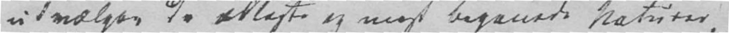udvælger de ædleste og mest begavde Naturer,
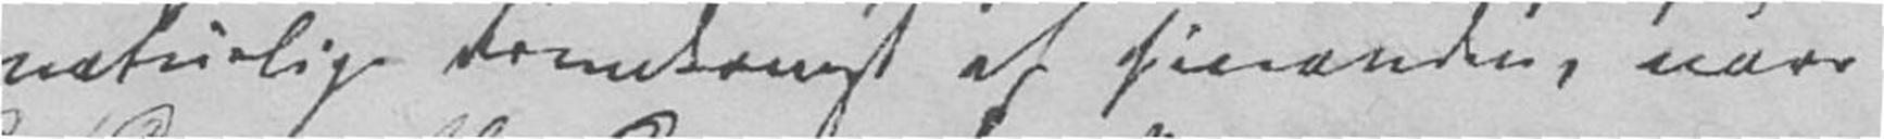naturlige Fremkomst af hinanden, naar
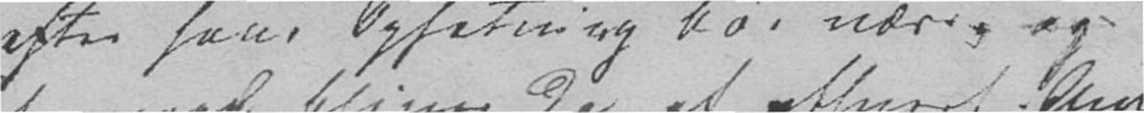efter hans Opfatning bør være, og
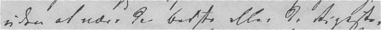uden at være de bedste eller de Rigeste,
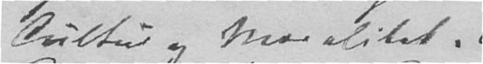Cultur og Moralitet.
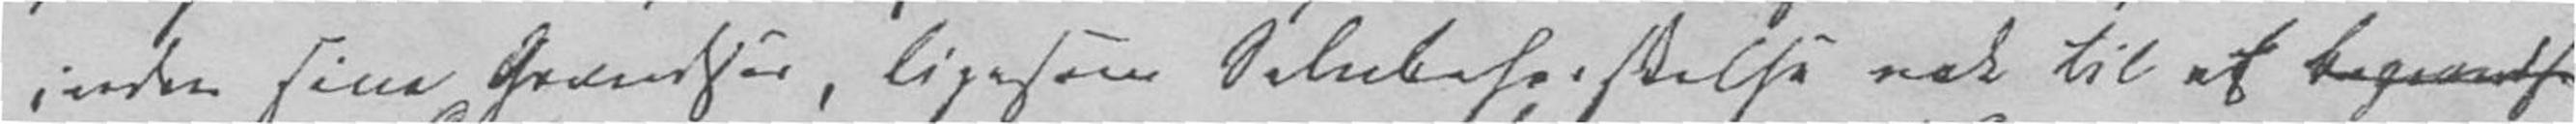inden sine Grændser, ligesom Selvbeherskelse nok til at
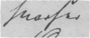hvorfor
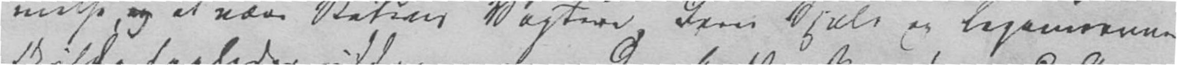melse, og at være Statens Vogtere. Deres Sjæle og Legemsevner
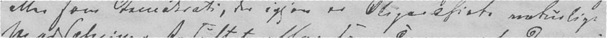eller som Demokrati, der igjen er Oligarchiets naturlige
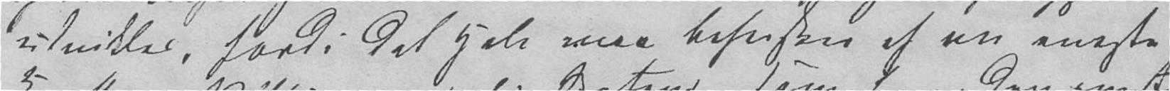udvikles, fordi det Hele maa beherskes af een eneste
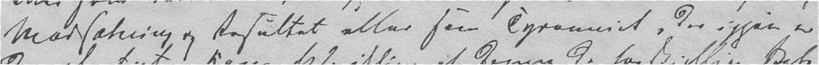Modsætning og Resultat eller som Tyranniet, der igjen er
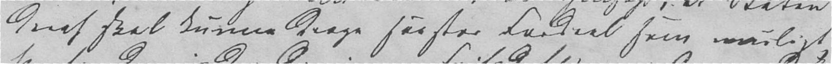deraf skal kunne drage saa stor Fordeel som muligt
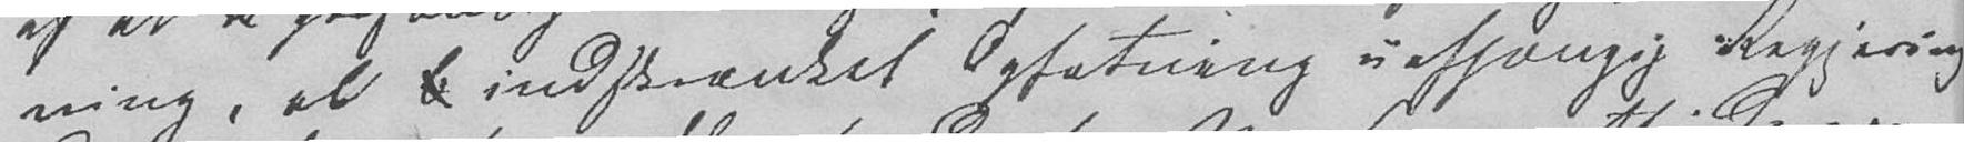ning, al b indskrænket Opfatning uafhængig Regjering.
